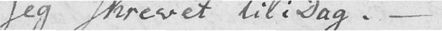jeg skrevet til i Dag. –
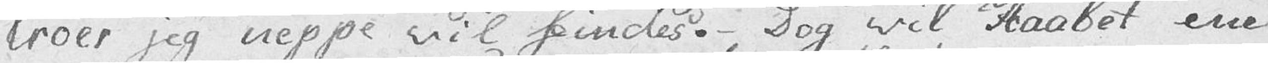troer jeg neppe vil findes. Dog vil Haabet ene
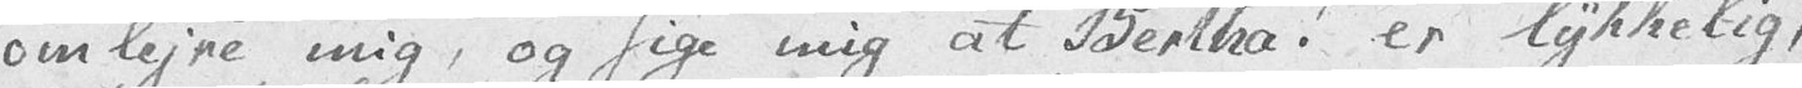omlejre mig, og sige mig at Bertha! er lykkelig,
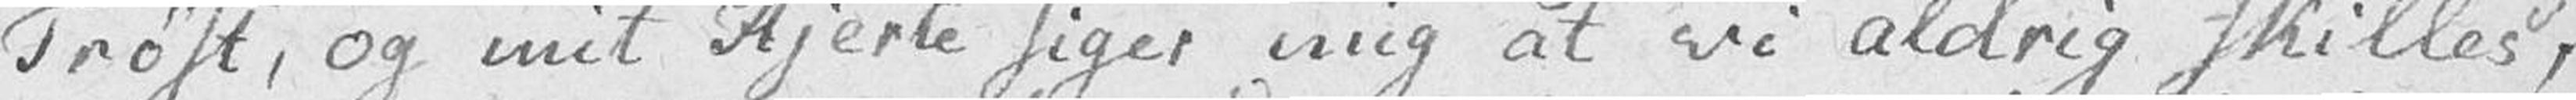Trøst, og mit Hjerte siger mig at vi aldrig skilles,
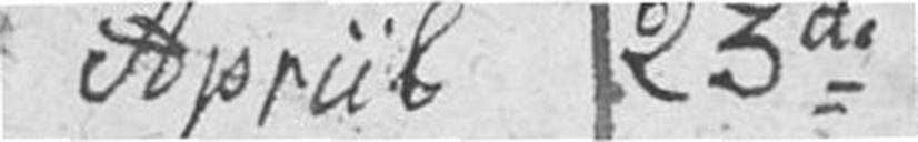Apriil 23de
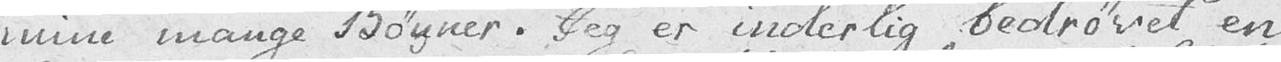mine mange Bønner. Jeg er inderlig bedrøvet en
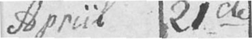Apriil 21de
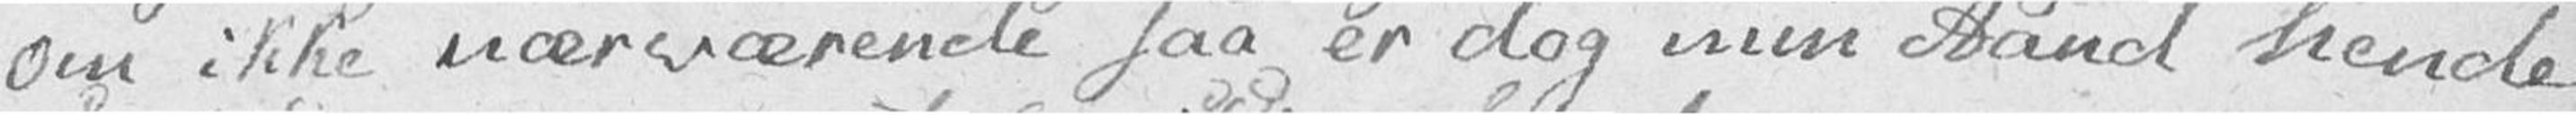om ikke nærværende saa er dog min Aand hende
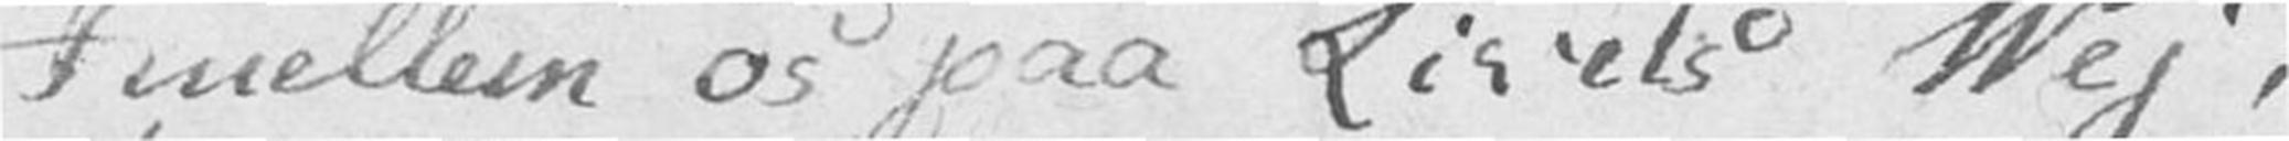I mellem os paa Livets Vej,
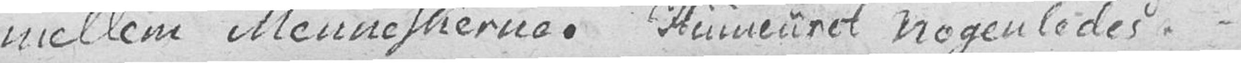mellem Menneskerne. Humeuret nogenledes. –
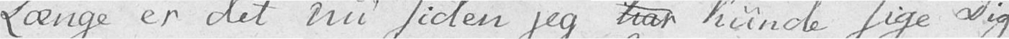Længe er det nu siden jeg har kunde sige Dig
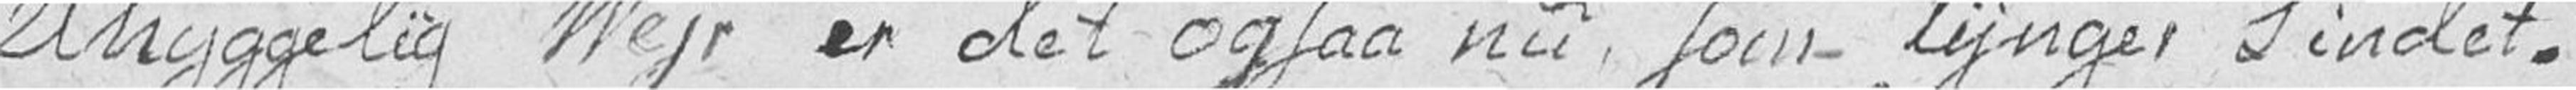Uhyggelig Vejr er det ogsaa nu, som tynger Sindet.
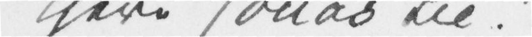Kjere Jonas Lie!
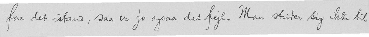faa det istand, saa er jo ogsaa det fejl. Man strider sig ikke til
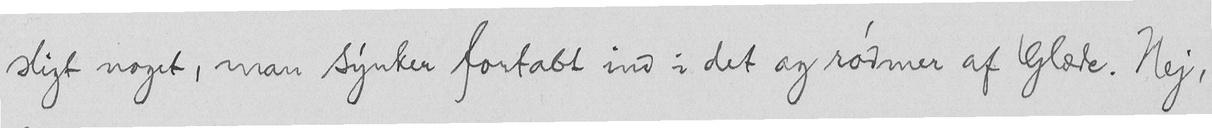sligt noget, man synker fortabt ind i det og rødmer af Glæde. Nej,
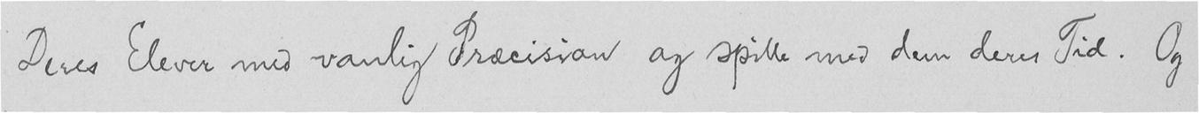Deres Elever med vanlig Præcision og spille med dem deres Tid. Og
